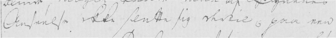Anseelse ikke slutte sig dertil; paa een
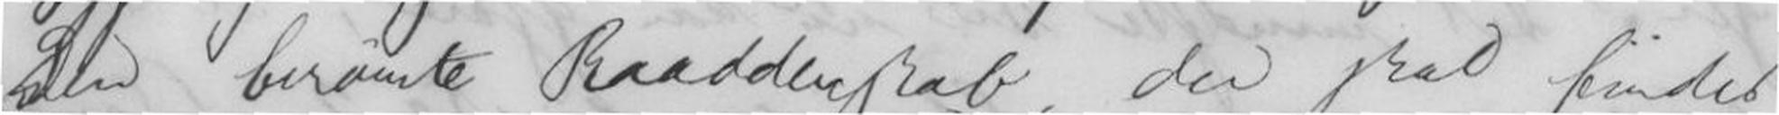Den berømte Raadderskab, der skal findet
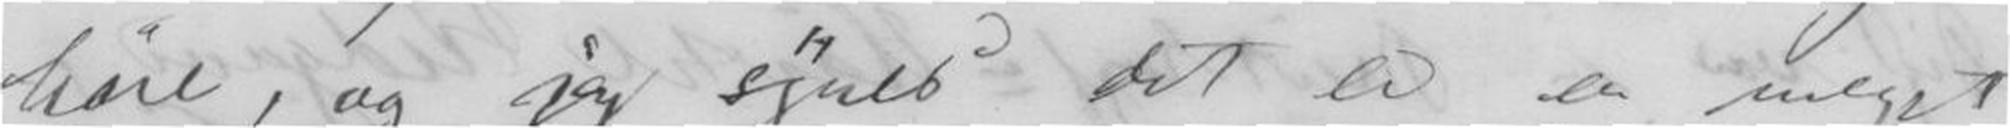høre, og jeg synes det er en meget
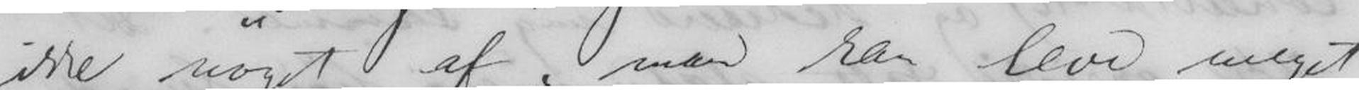ikke noget af; man kan leve meget
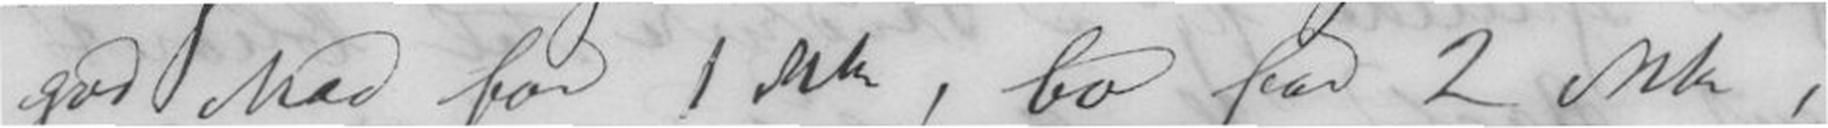god Mad for 1 Mk, bo for 2 Mk,
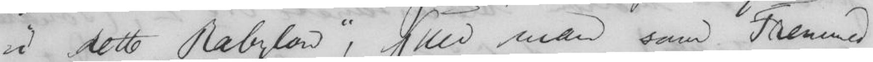i dette Babylon, skue man som Fremmed
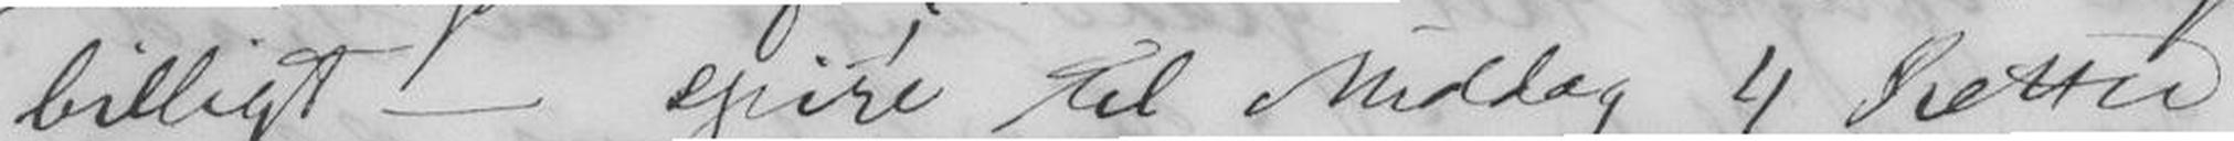billigt - spise til Middag 4 Retter
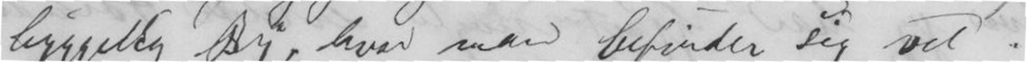hyggelig By, hvor man befinder sig vel.
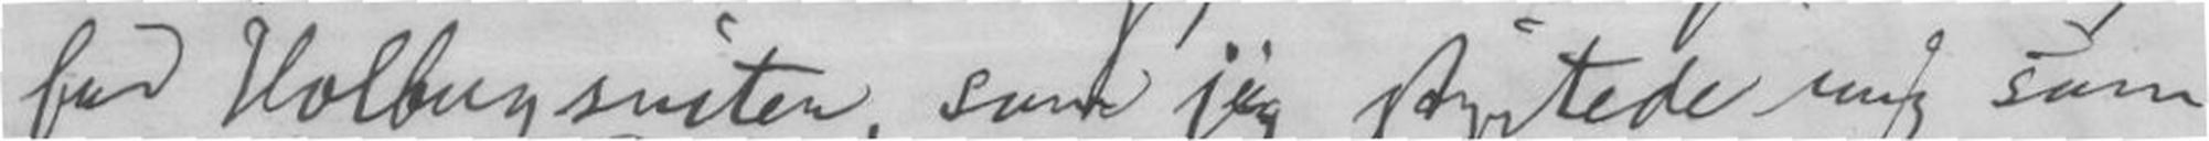for Hobergsuiten, som jeg styrtede mig som
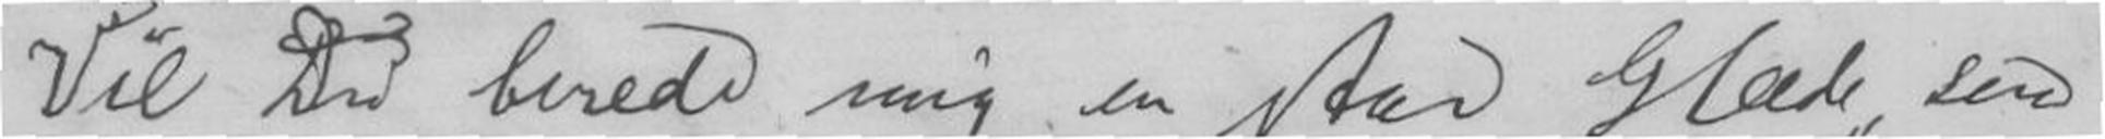Vil Du berede mig en stor Glæde, saa
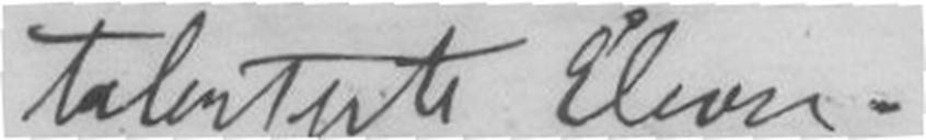talenterte Elever.
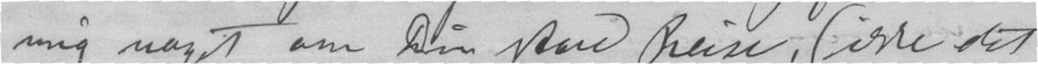mig noget om Din store Reise, (ikke det
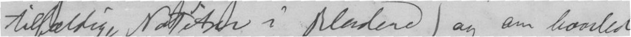tilfældige Notiser i Bladene) og om hvorledes
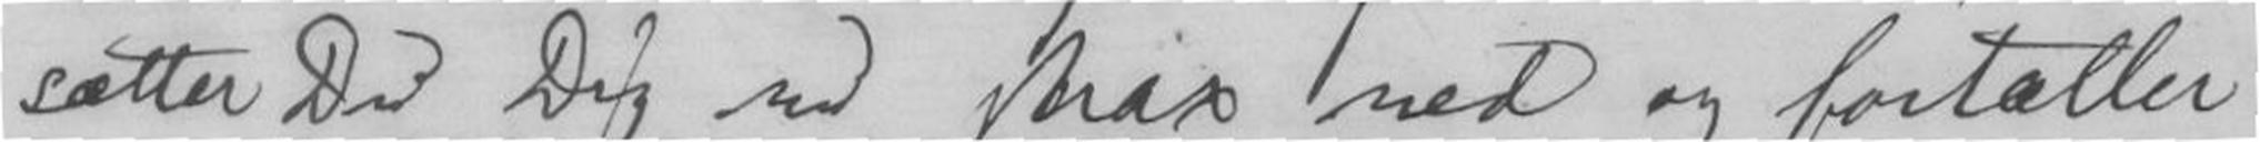sætter Du Dig strax ned og fortæller
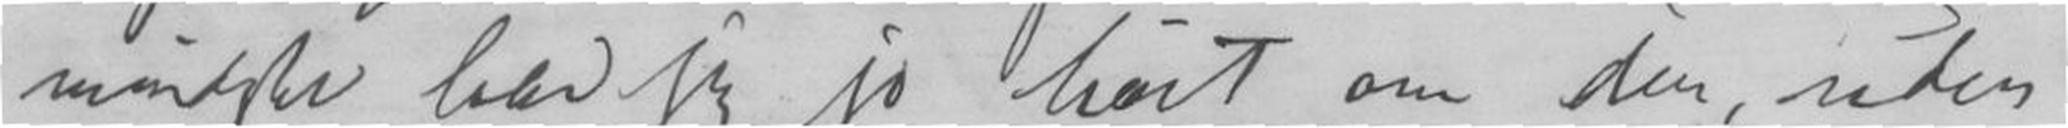mindste har jeg jo hørt om den, uden
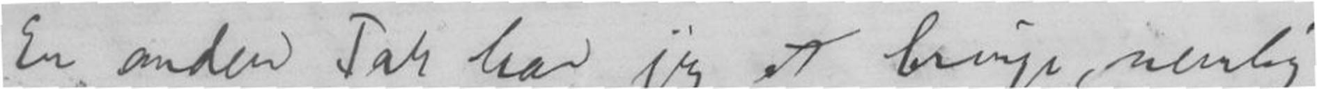En anden Tak har jeg at bringe, nemlig
